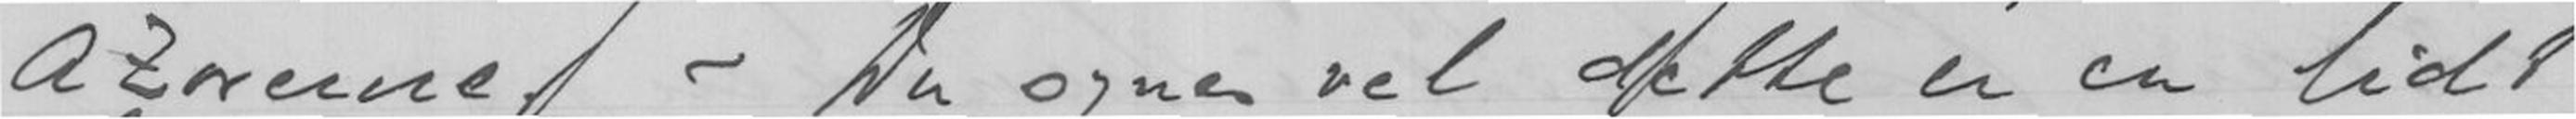Azorene - Du synes vel dette er en lidt
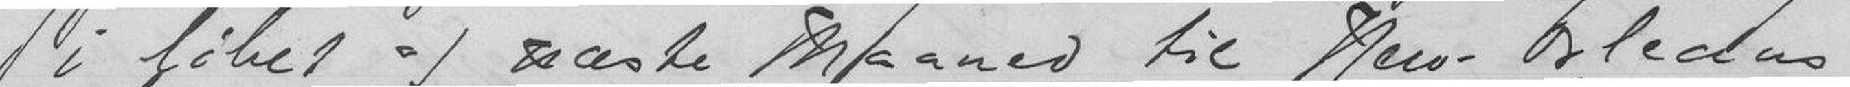i løbet af næste Maaned til New-Orleans
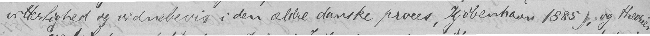vitterlighed og vidnebevis i den ældre danske proces, Kjøbenhavn 1885), og theorien
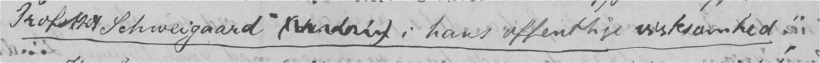"Professor Schweigaard  i hans offentlige virksomhed",
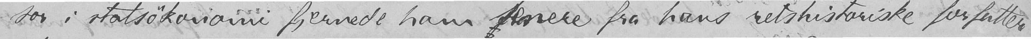sor i statsøkonomi fjernede ham senere fra hans retshistoriske forfatter-
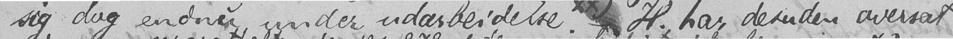sig dog endnu under udarbeidelse. xx) H. har desuden oversat
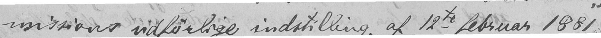missions udførlige indstilling, af 12te februar 1881,
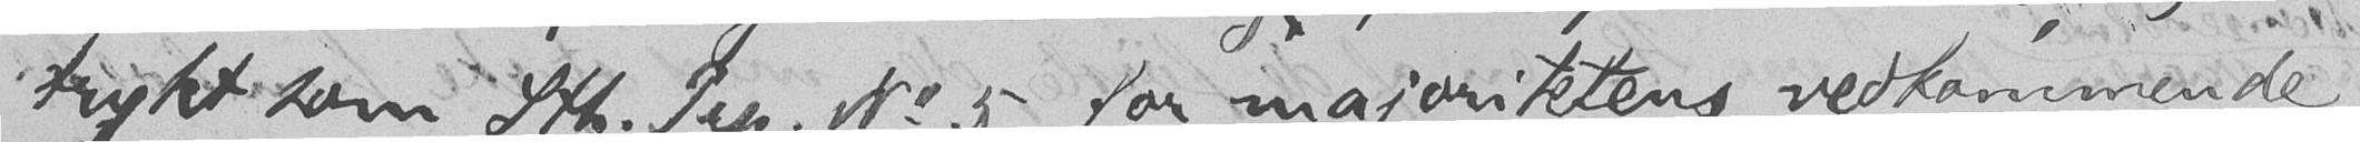trykt som Sth. Prp. No 5, for majoritetens vedkommende
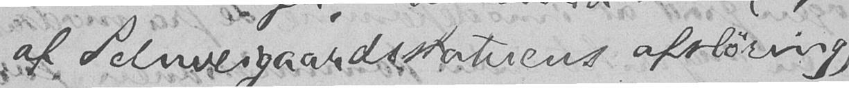af Schweigaardstatuens afsløring)
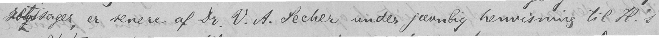retssager, er senere af Dr. V. A. Secher under jævnlig henvisning til H.'s
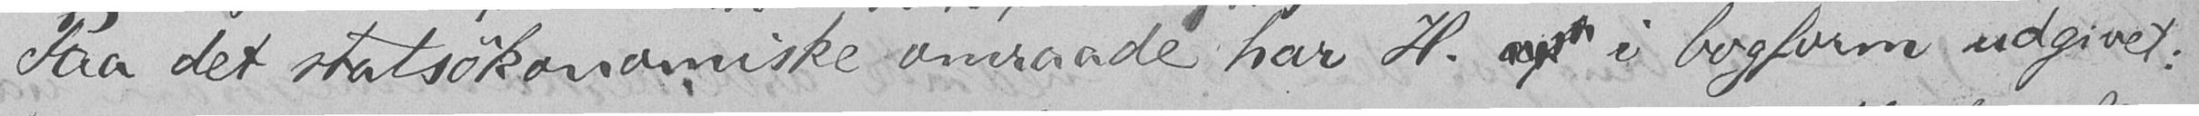Paa det statsøkonomiske omraade har H.  i bogform udgivet:
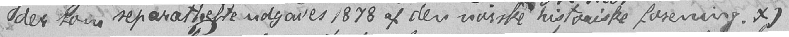der som separathefte udgaves 1878 af den norske historiske forening. x)
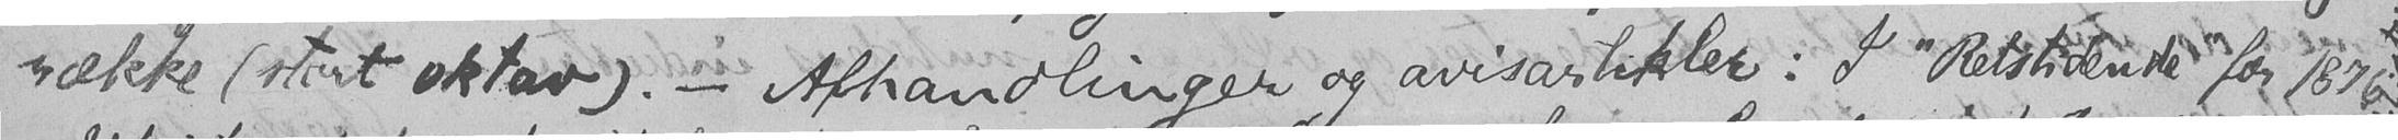række (stort oktav). - Afhandlinger og avisartikler: I "Retstidende" for 1876
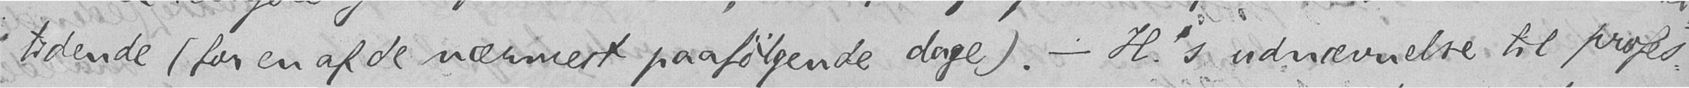tidende (for en af de nærmest paafølgende dage). - H.'s udnævnelse til profes-
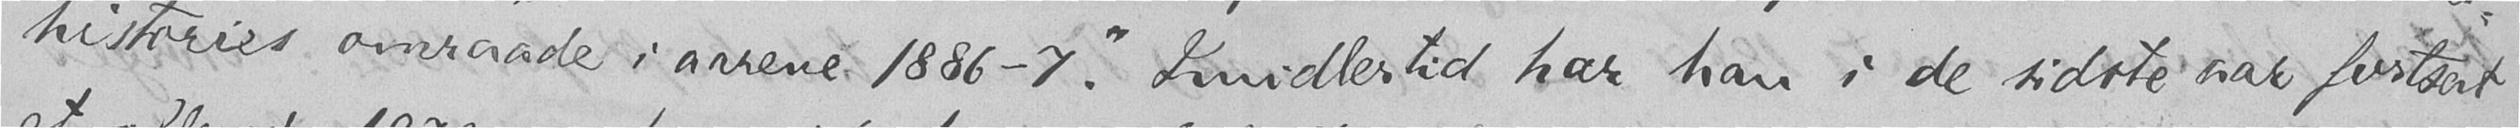histories omraade i aarene 1886-7." Imidlertid har han i de sidste aar fortsat
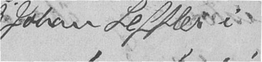Johan Leffler i
